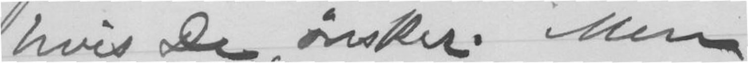hvis De ønsker. Men
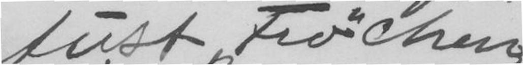Just Frøchen
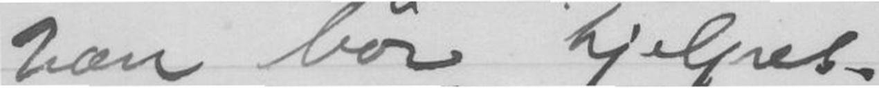han bør hjelpes.
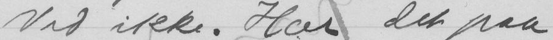Ved ikke. Har det paa
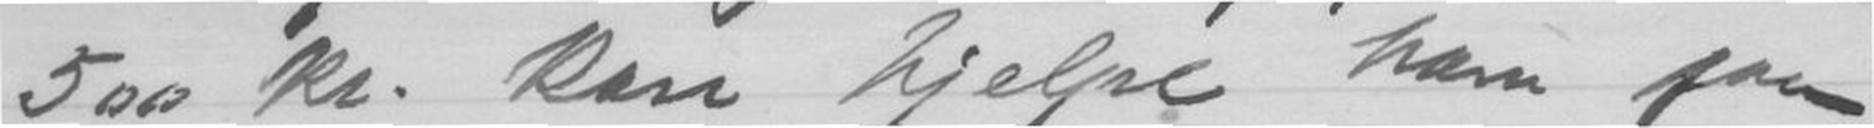500 kr. kan hjelpe ham paa
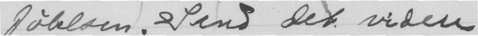følelsen. Send det videre,
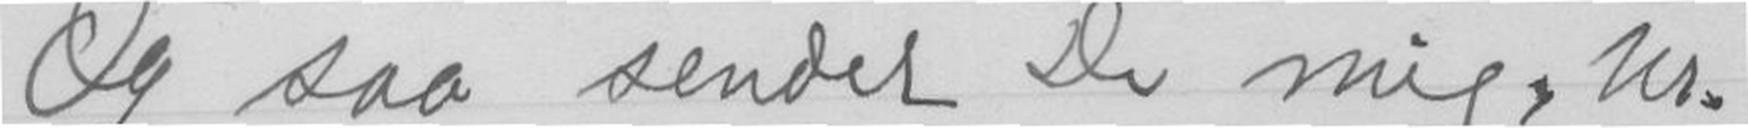Og saa sender De mig. Hr-
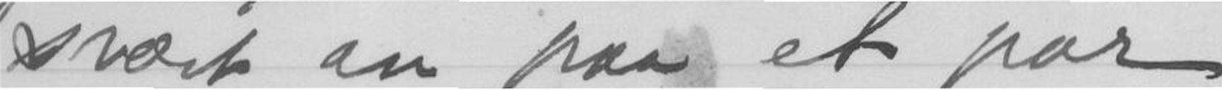svært an paa et par
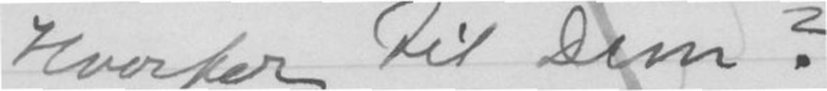Hvorfor til Dem?
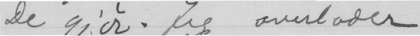De gjør. Jeg overlader
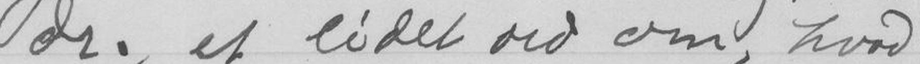dr; et lidet ord om, hvad
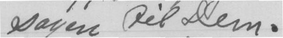sagen til Dem.
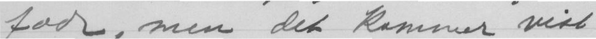fode, men det kommer vist
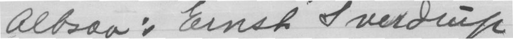Altsaa: Ernst Sverdrup
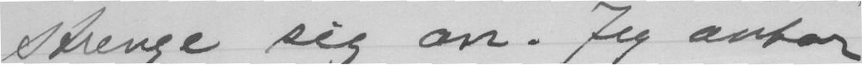strenge sig an. Jeg antar
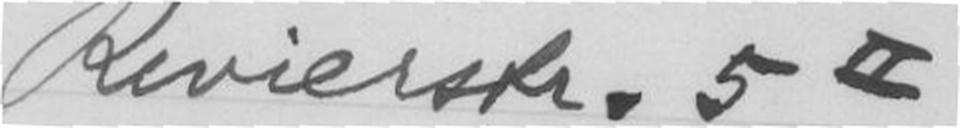Rivierstr. 5II
